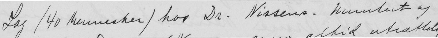Lag (40 Mennesker) hos Dr. Nissens. Muntert og
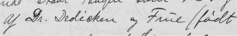af Dr. Dedicken og Frue (født
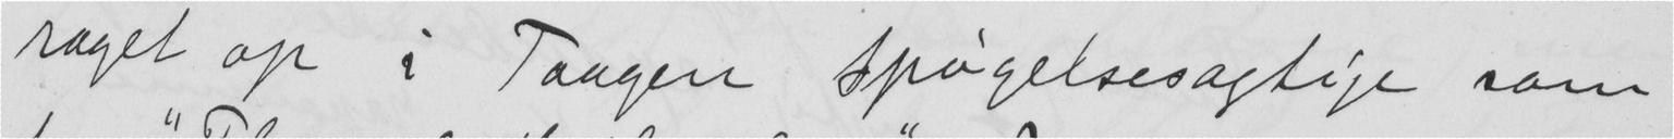raget op i Taagen spøgelsesagtige som
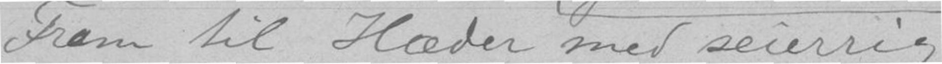Fram til Hæder med seierrig
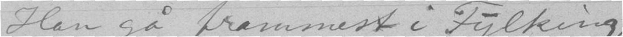Han gå frammest i Fylking,
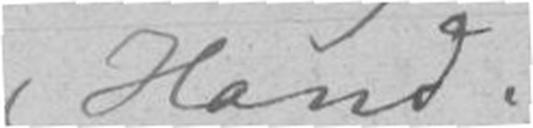Hand.
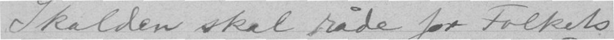Skalden skal råde for Folkets
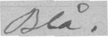Blå.
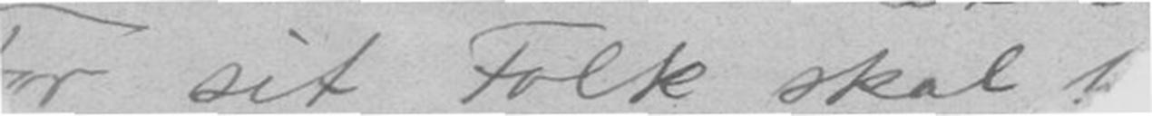For sit Folk skal
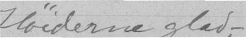Høiderne glad, -
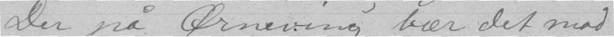Der på Ørneving bær det mod
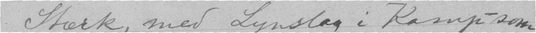Stærk, med Lynslag i Kamp - som
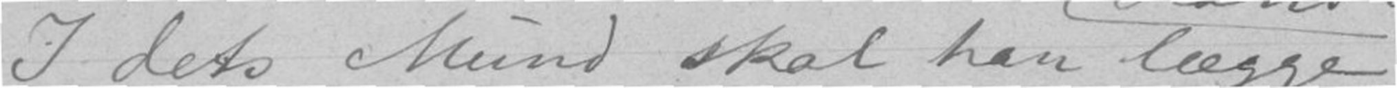I dets Mund skal han lægge
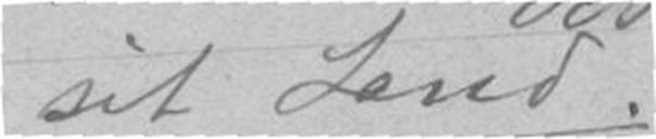sit Land.
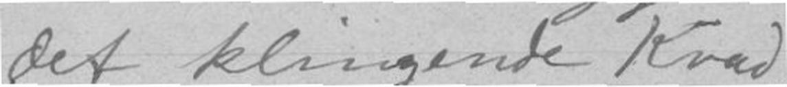det klingende Kvad,
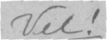Vel!
